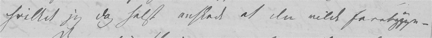hvilket jeg dog helst ønskede at Du vilde forebygge –
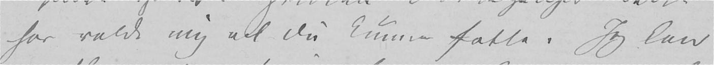har voldt mig vil Du kunne fatte. Jeg kan
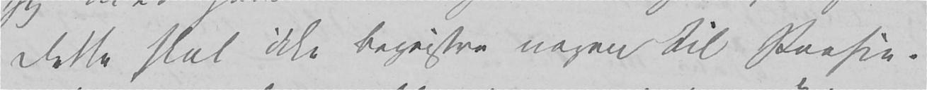Dette skal ikke begeistre nogen til Poesie.
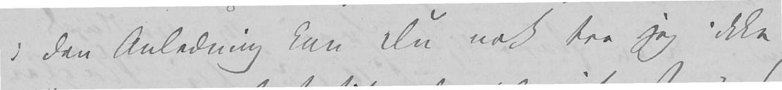i den Anledning kan Du nok tro jeg ikke
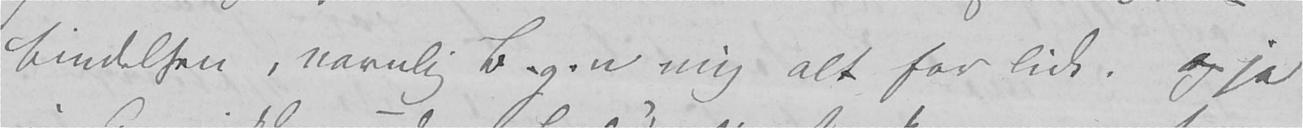bindelsen, navnlig B.g.n mig alt for lidt, og ja
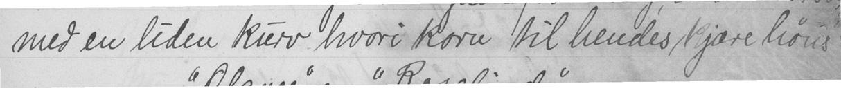med en liden kurv hvori korn til hendes kjære høns
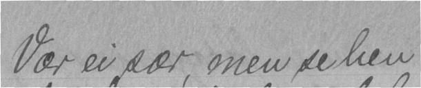Vær ei sær, men se hen
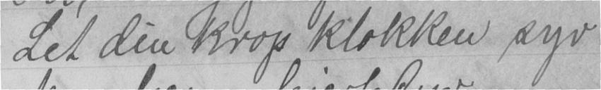Let din krop klokken syv
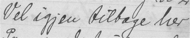Vel igjen tilbage her
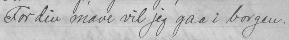For din mave vil jeg gaa i borgen.
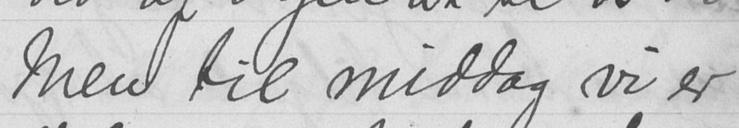Men til middag vi er
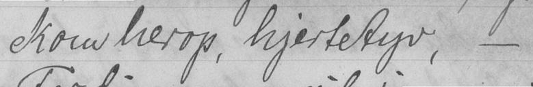Kom herop, hjertetyv, -
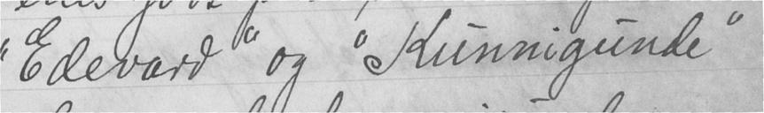"Edevard", og "Kunnigunde"
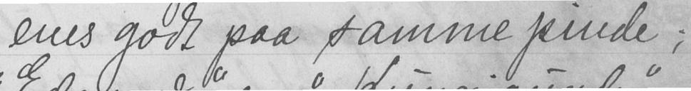enes godt paa samme pinde;
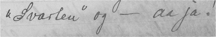"Svarten" og - aa ja!
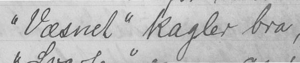"Væsnet" kagler bra,
